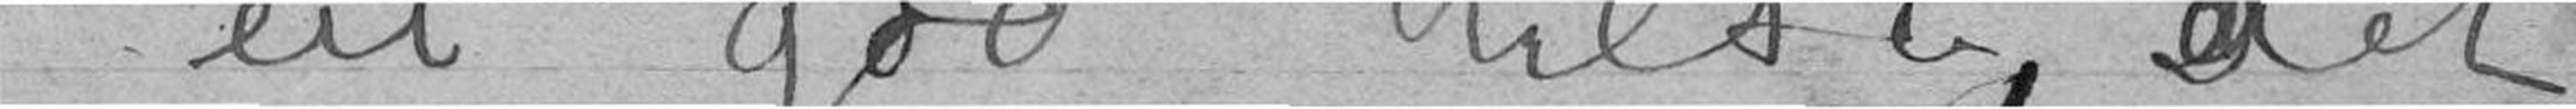en god helse; det
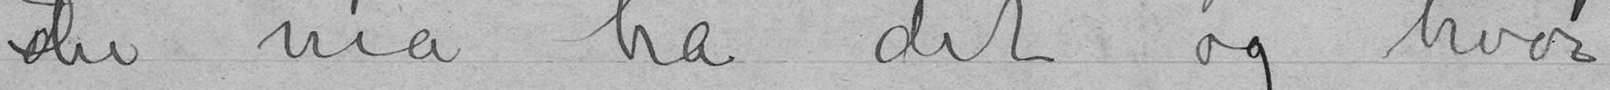Du må ha det og hvor
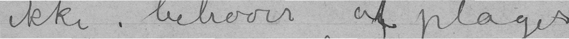ikke behøver at plages
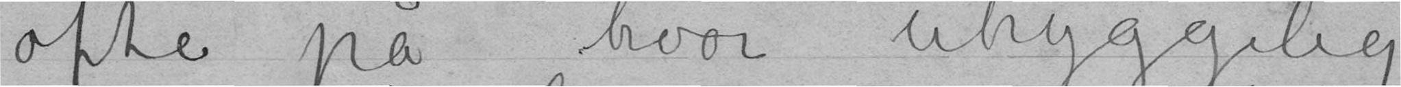ofte på hvor uhyggelig
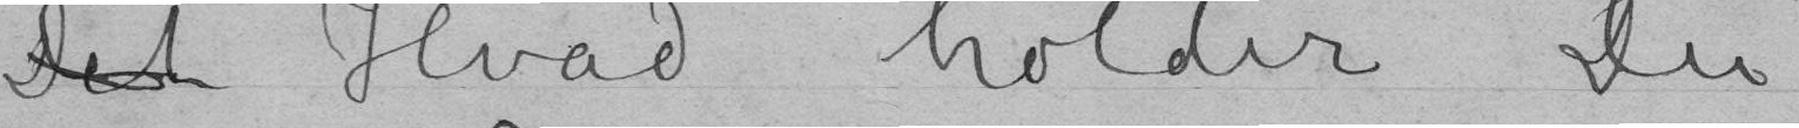Det Hvad holder Du
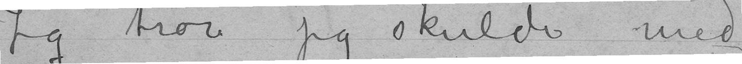Jeg tror jeg skulde med
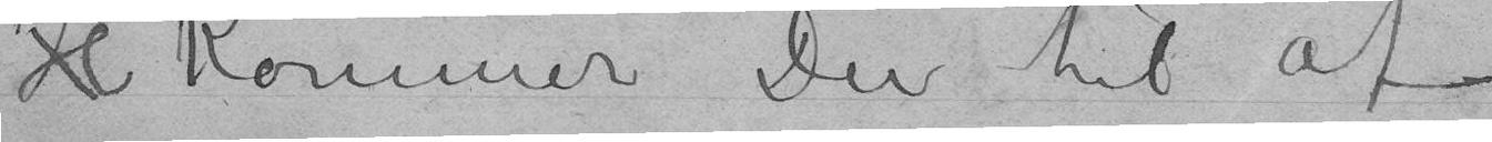H Kommer Du til at
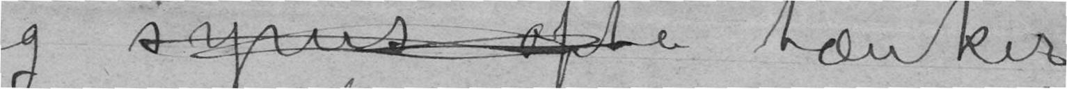Jeg synes oft tænker
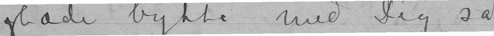glæde bytte med Dig så
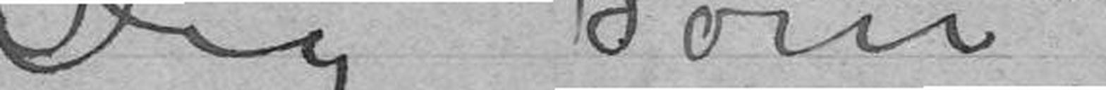Dig som
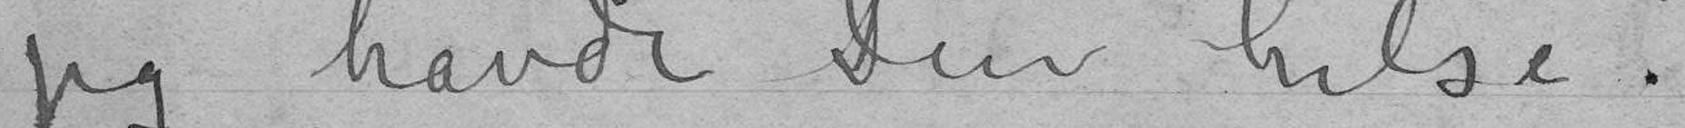jeg havde Din helse.
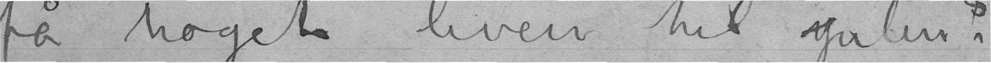få noget leven til julen?
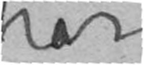har
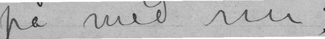på med nu?
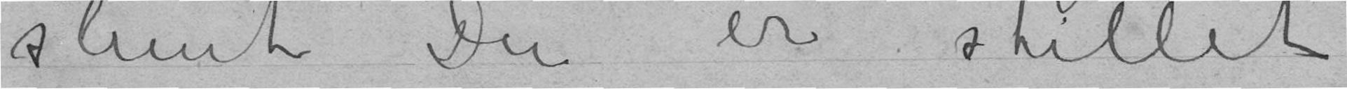slemt Du er stillet
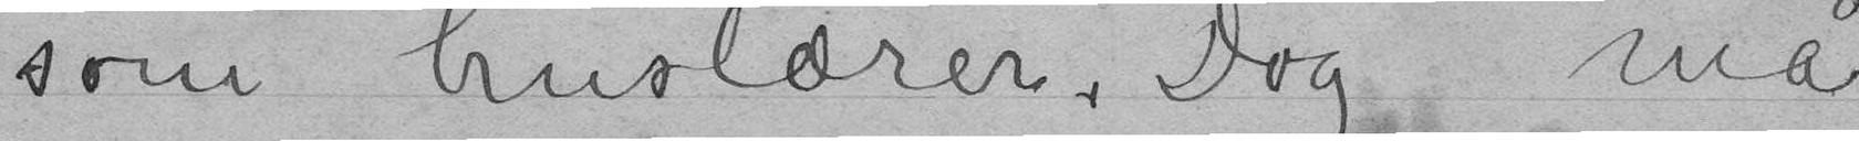som huslærer. Dog må
